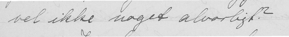vel ikke noget alvorligt?
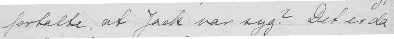fortalte, at Jack var syg? Det er da
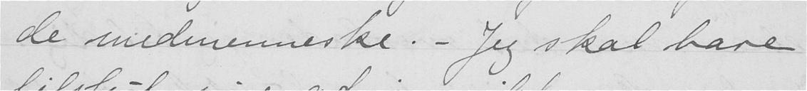de medmennske. - Jeg skal bare
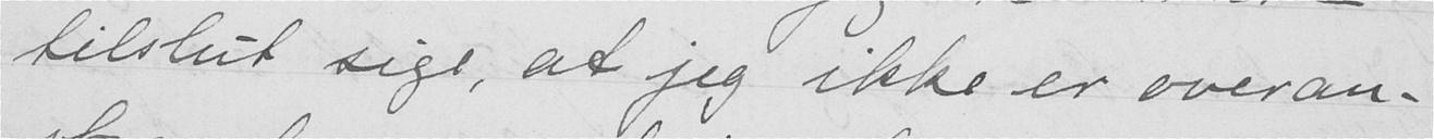tilslut sige, at jeg ikke er overan-
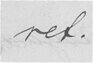ret.
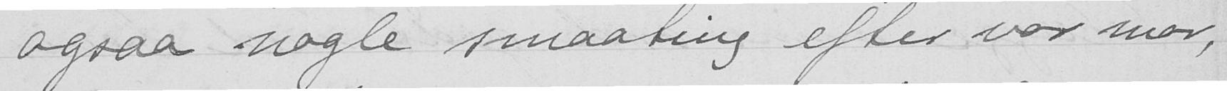ogsaa nogle smaating efter vor mor,
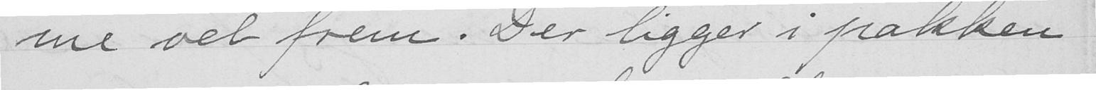me vel frem. Der ligger i pakken
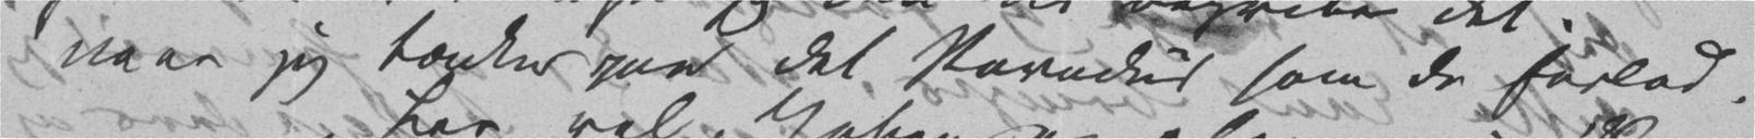naar jeg tænker paa det Paradiis som de forlod.
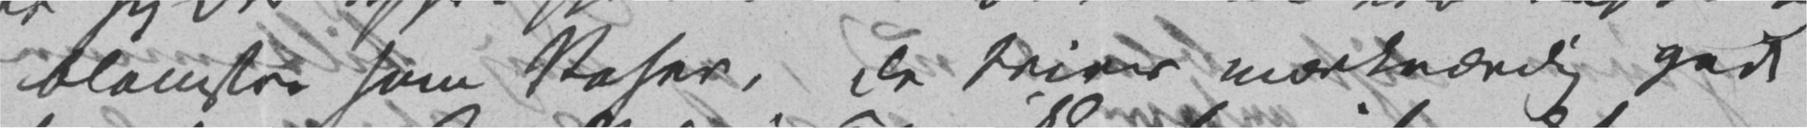blomstre som Roser, de trives mærkværdig godt
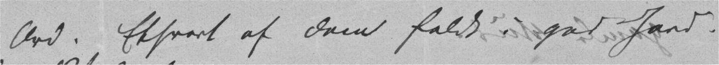Ord. Ethvert af dem faldt i god Jord.
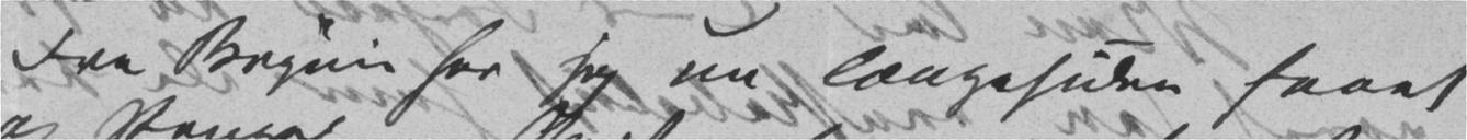Fra Brynie har jeg nu længesiden faaet
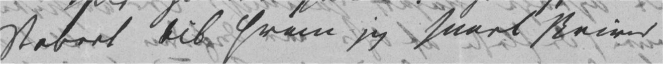Robert til hvem jeg snart skriver.
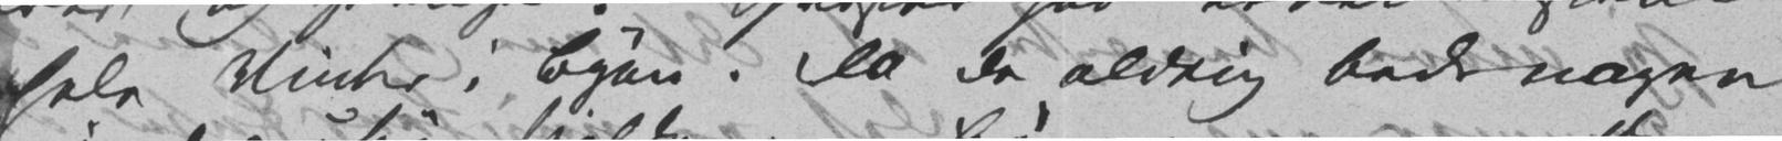hele Vinter i Byen. Da de aldrig bede nogen,
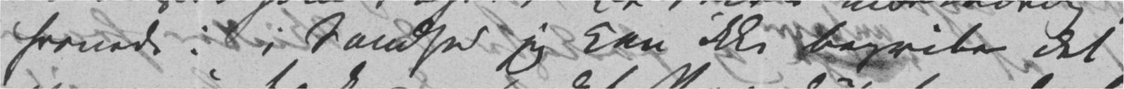hernede: i Sandhed jeg kan ikke begribe det,
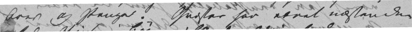Brev og Penge. Qvæstor har været næsten den
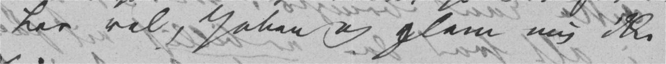Lev vel, Johan og glem mig ikke
